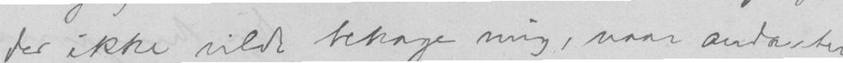der ikke vilde behage mig, naar andanten
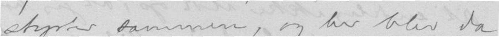styrter sammen, og her blir da -
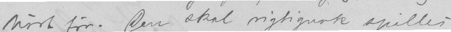hørt før. Den skal rigtignok spilles
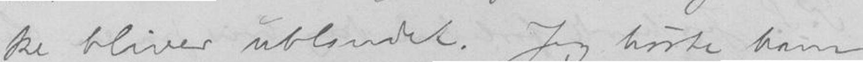ke bliver ublandet. Jeg hørte ham
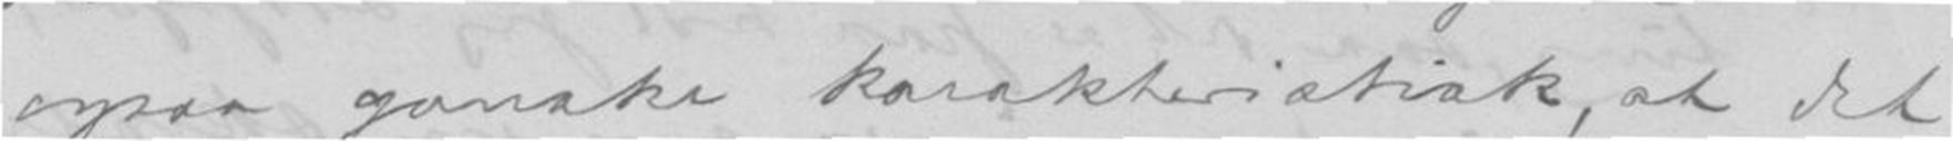ogsaa ganske karakteristisk, at det
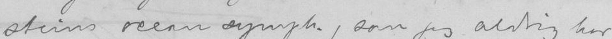steins ocean symph., som jeg aldrig har
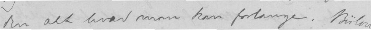den alt hvad man kan forlange. Bülow
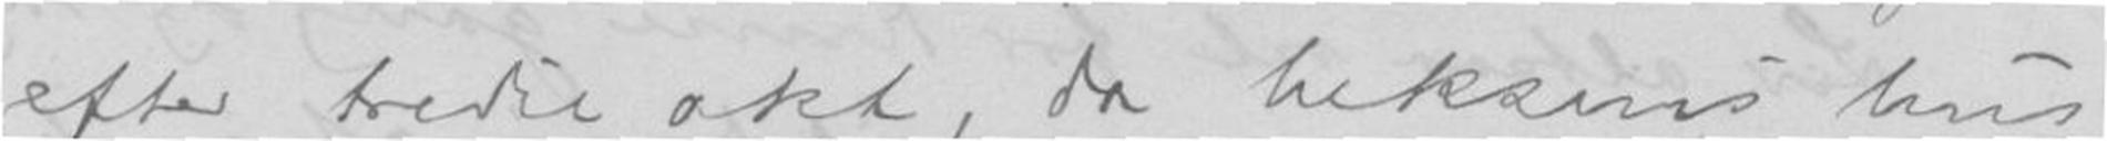efter tredje akt, da heksens hus
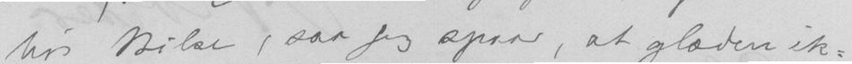hos Bilse, saa jeg spaar, at glæden ik-
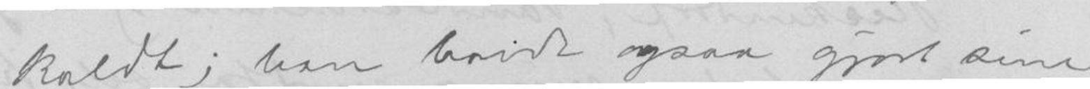kaldt, han havde ogsaa gjort sine
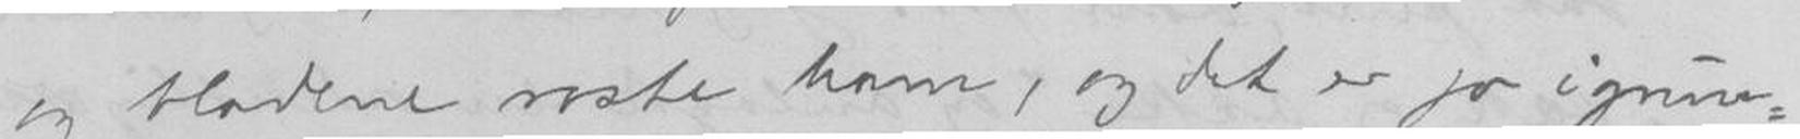og bladene roste ham, og det er jo igrunn-
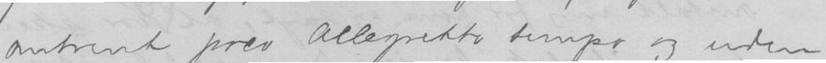omtrent preo Allegretto tempo og uden
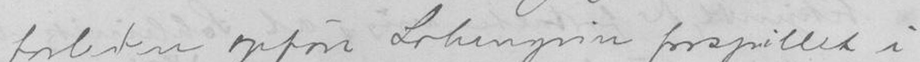forleden opføre Lohengrin forspillet i
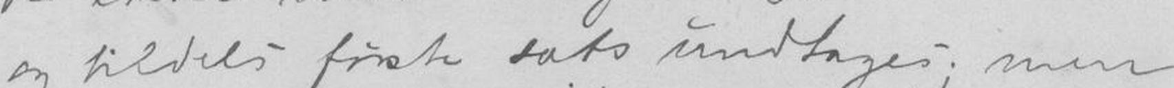og tildels første sats undtages; men
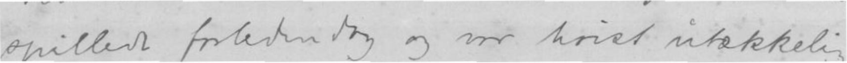spillede forleden dog og var høist utækkelig,
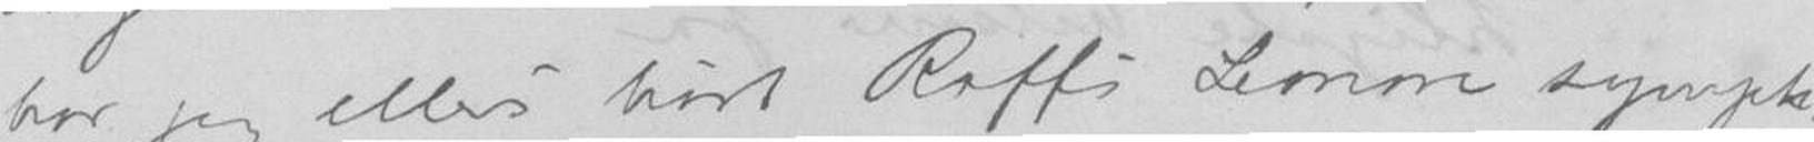har jeg ellers hørt Roffi Leonori symph.
